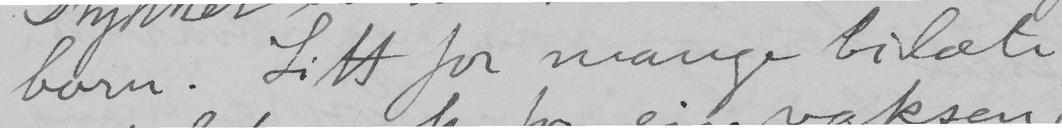born. Litt for mange bilæte
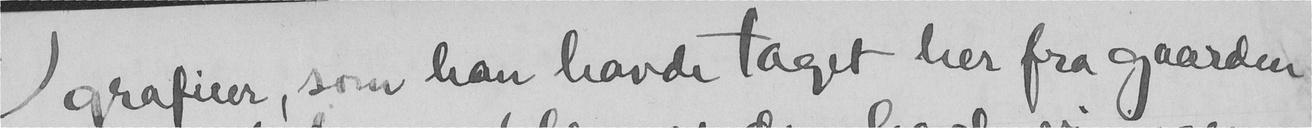grafieer, som han havde taget her fra gaarden
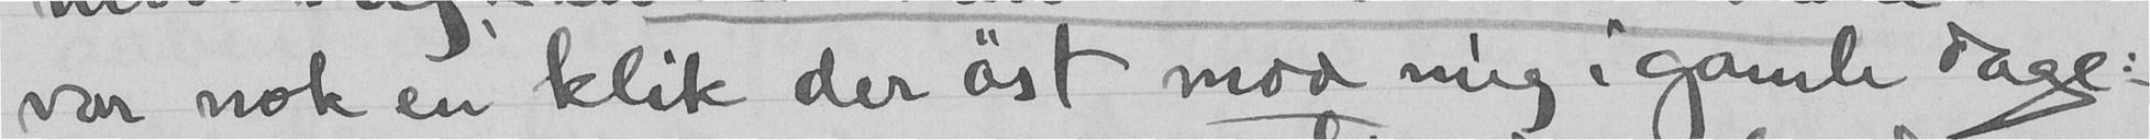var nok en klik der øst mod mig i gamle dage:
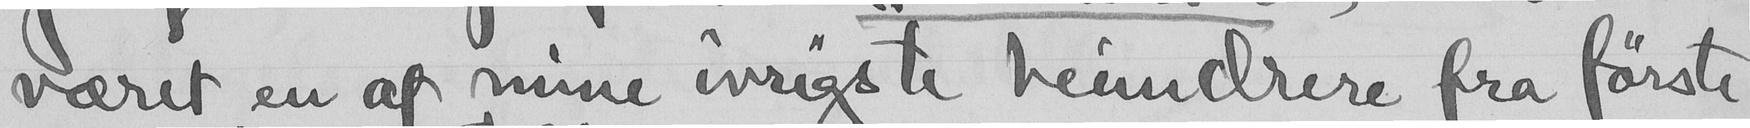været en af mine ivrigste beundrere fra første
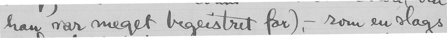han var meget begeistret for), - som en slags
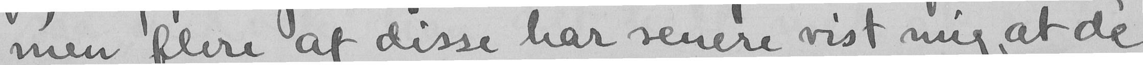men flere af disse har senere vist mig, at de
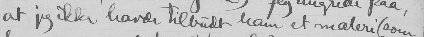at jeg ikke havde tilbudt ham et maleri (som
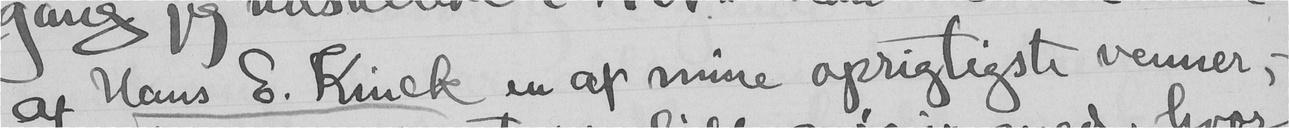af Hans E. Kinck en af min oprigtigste venner,-
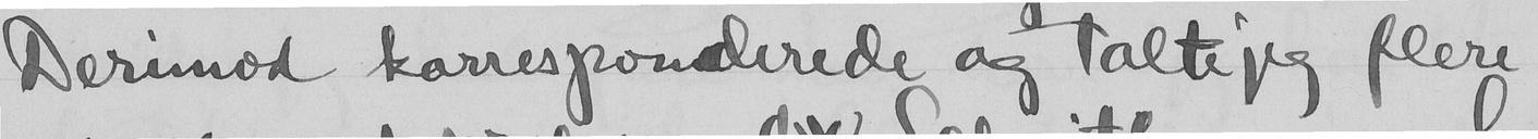Derimod korresponderede og talte jeg flere
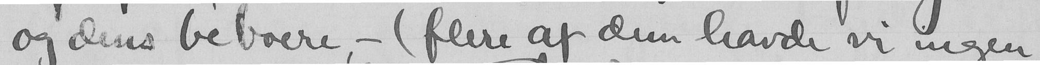og dens beboere - (flere af dem havde vi ingen
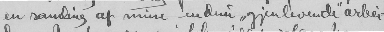en samling af mine endnu "gjenlevende arbei-
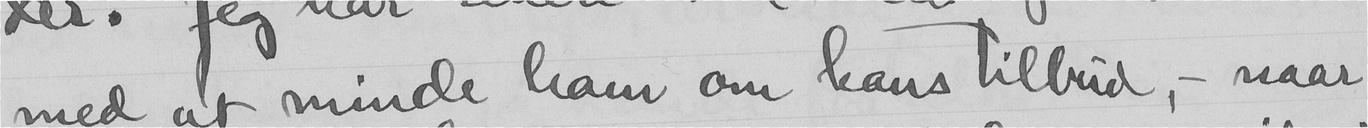med at minde ham om hans tilbud, - naar
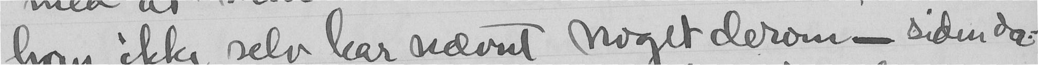han ikke selv har nævnt noget derom - siden da.-
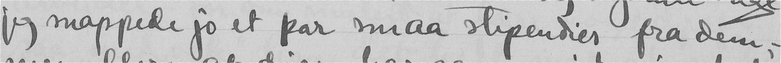jeg mappede jo et par smaa stipendier fra dem,-
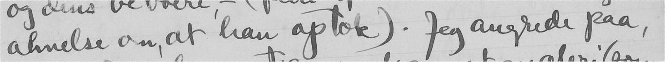ahnelse om, at han optok). Jeg angrede paa,
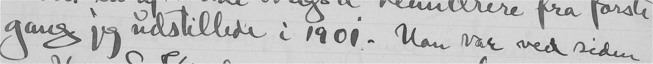gang jeg udstillede i 1901. Han var ved siden
